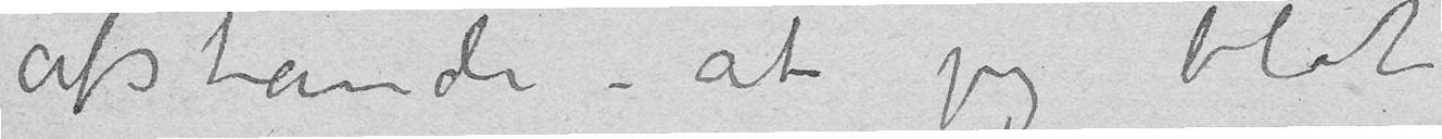afstande – at jeg blot
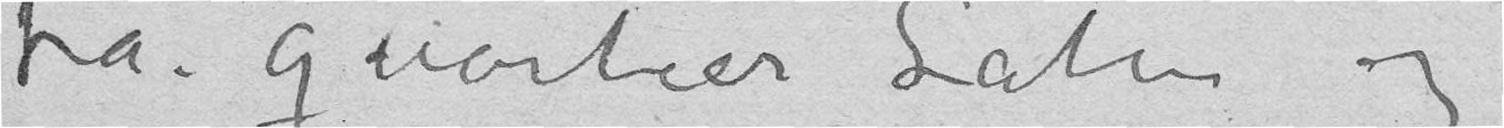fra quartier Latin og
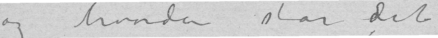og hvordan står det
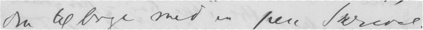den tilbage med en pen Skrevel-
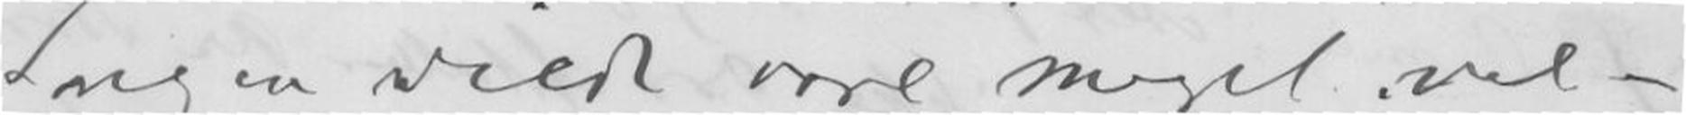Sangen vilde være meget vel-
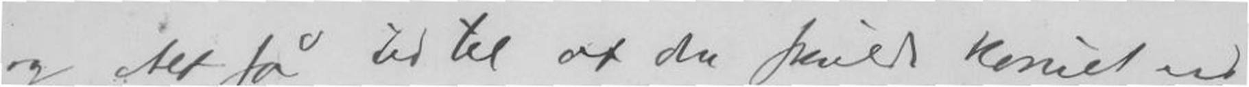og Altså tid til at du skulde Komet ud
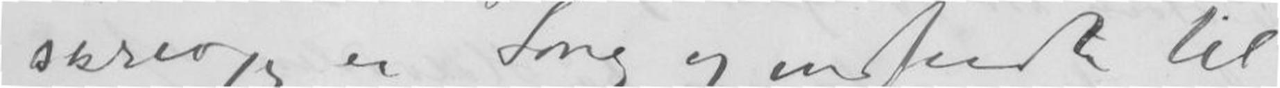skrev jeg en Sang og er sendt til
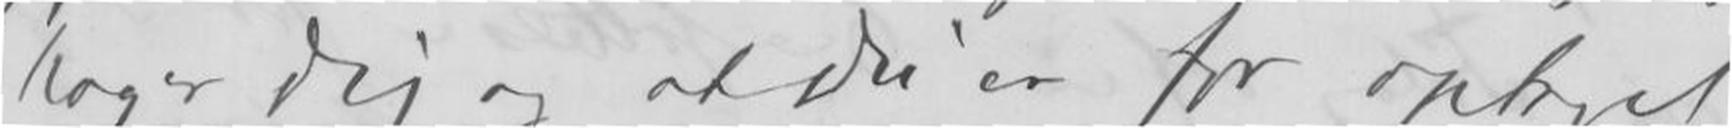hager dig og at du er for optaget
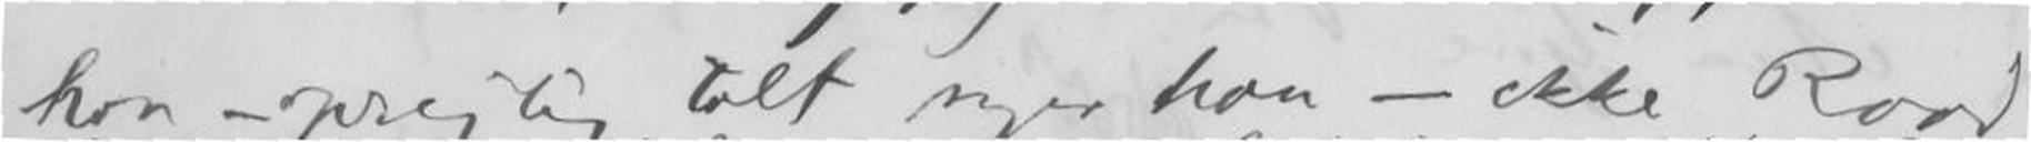han - oprigtig talt siger han - ikke Raad
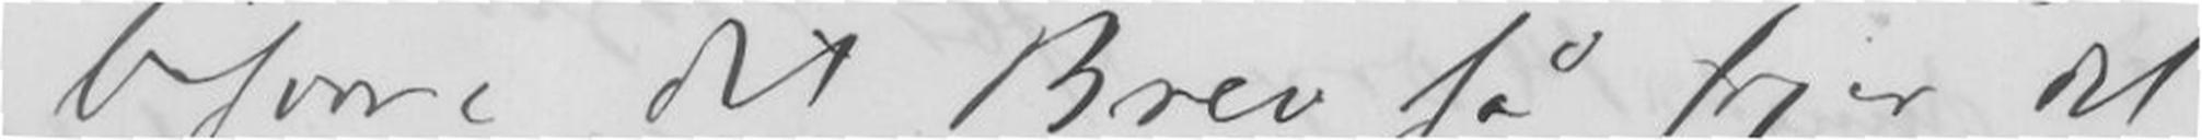besvare dit Brev så siger det
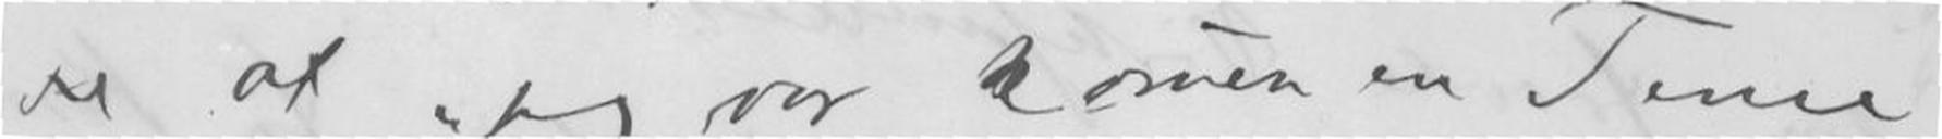e at jeg var komen en Time
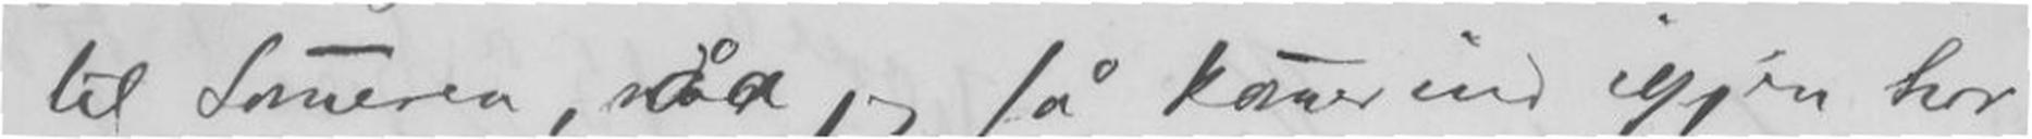til Someren, må jeg få kome ind igjen tror
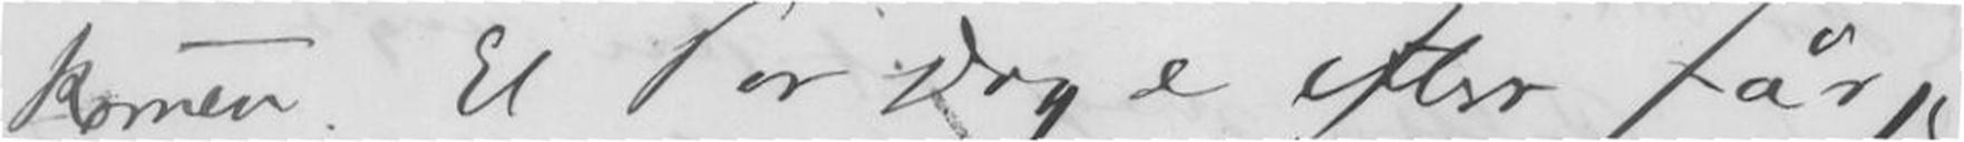omen. Et Par dage efter får jeg
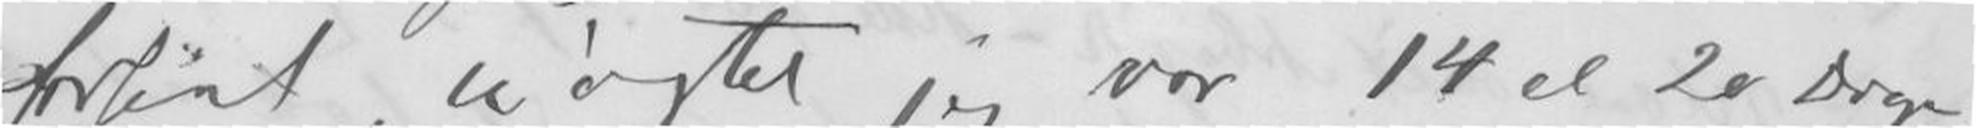forsent nægtet jeg var 14 el 20 Dage
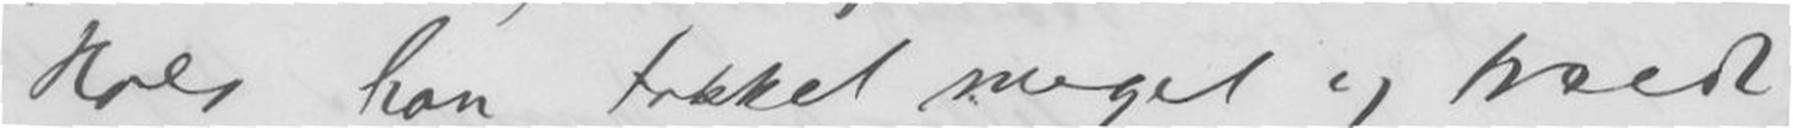Hals han takket meget og troede
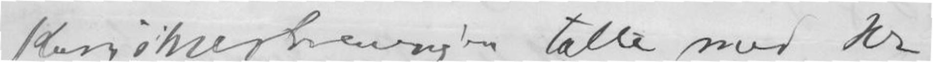Kunstnerforeningen talte med Hr
